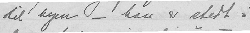til byen - han er stedt i
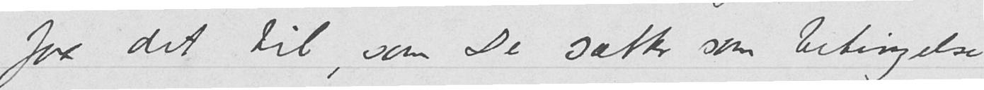faa det til, som De sætter som betingelse
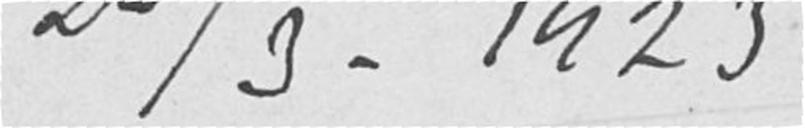20/3-1923
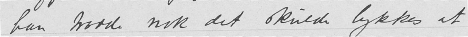- han trodde nok det skulde lykkes at
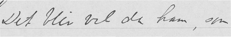Det blir vel da ham, som
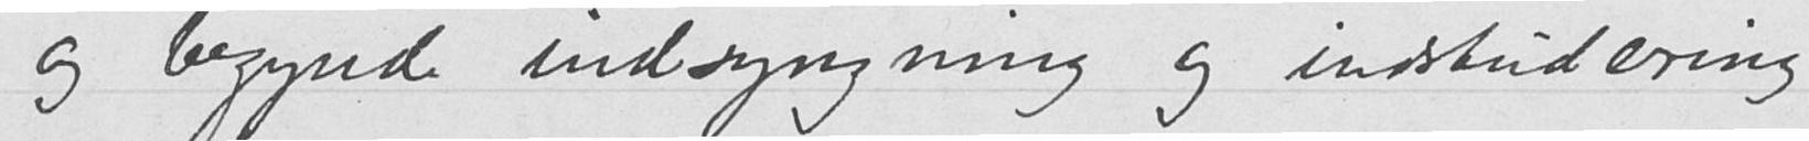og begynde indsyngning og indstudering
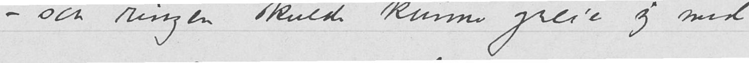– saa ringen skulde kunne greie sig med
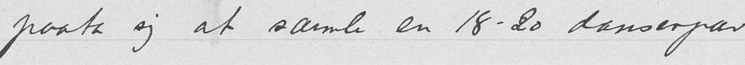paata sig at samle en 18-20 danserpar
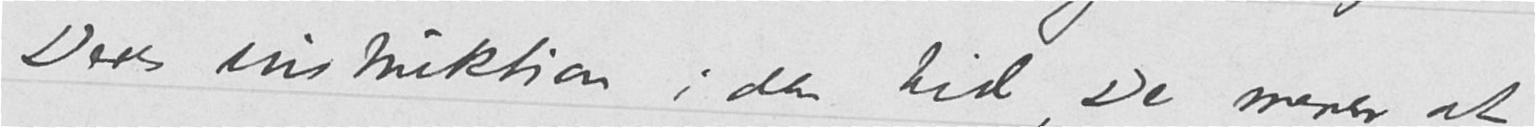Deres instruktion i den tid, De mener at
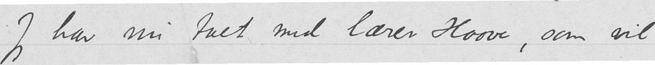Jeg har nu talt med lærer Haave, som vil
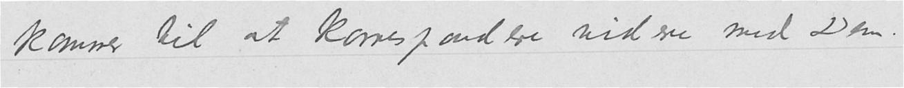kommer til at korrespondere videre med Dem.
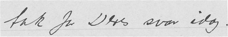tak for Deres svar idag.
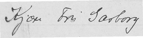Kjære Fru Garborg
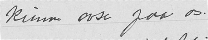kunne avse paa os.
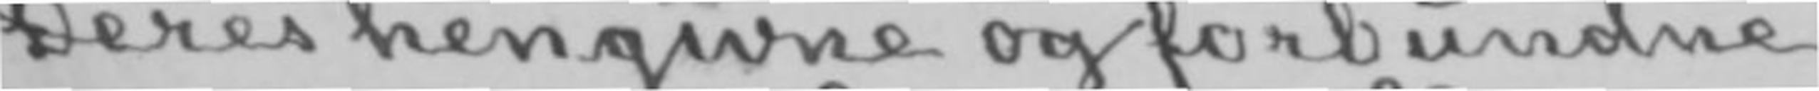Deres hengivne og forbundne
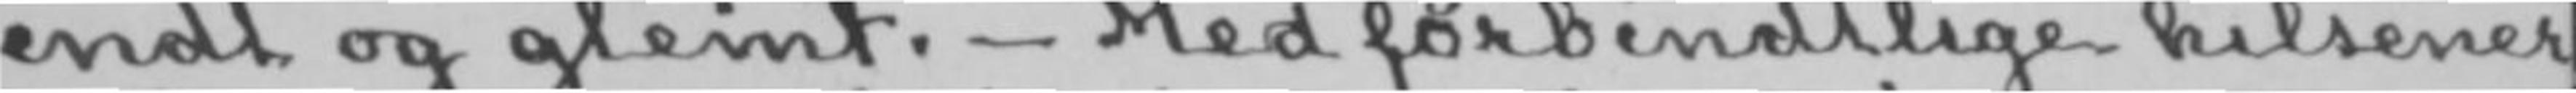endt og glemt.- Med forbindtlige hilsener
